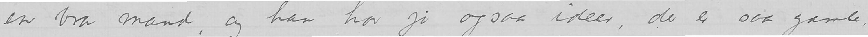en bra mand, og han har jo ogsaa ideer, der er saa gamle,
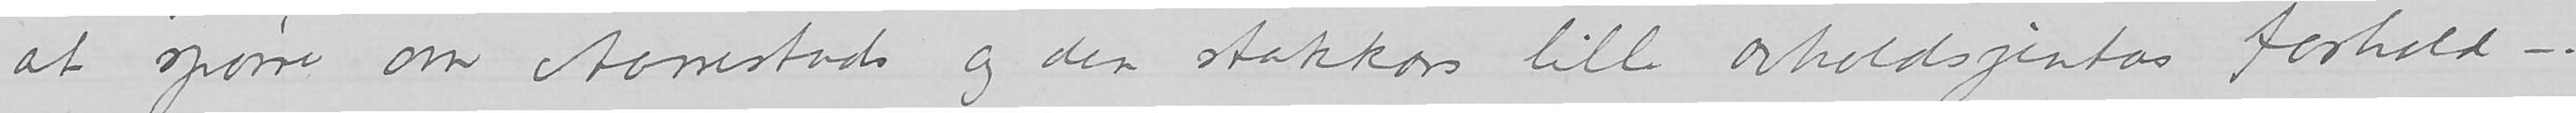at spørre om Aarrestads og den stakkars lille avholdsjentas forhold –.
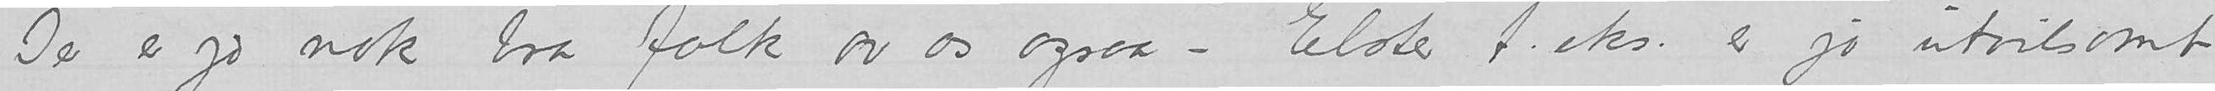Der er jo nok bra folk av os ogsaa – Elster f.eks. er jo utvilsomt
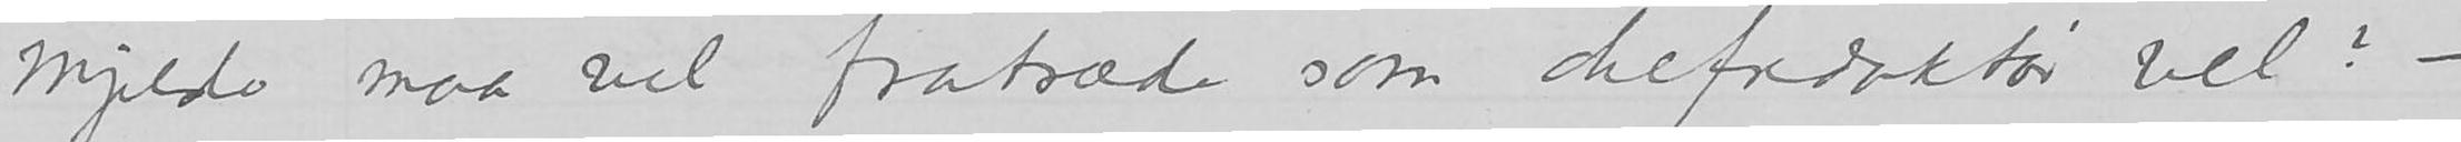Mjelde maa vel fratræde som chefredaktør vel? –
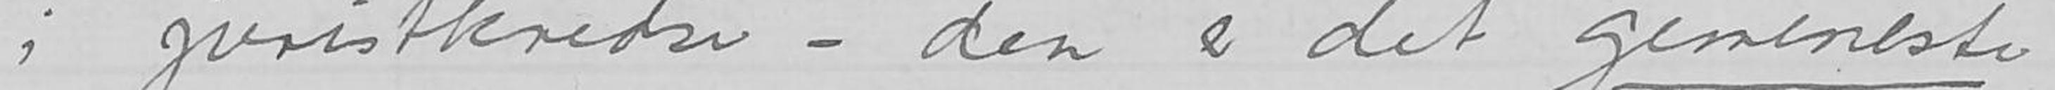i juristkredser – den er det gemeneste
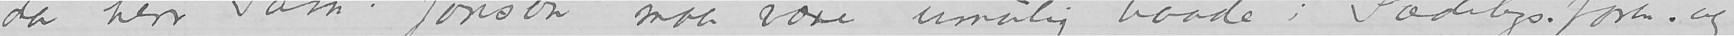da herr Sam. Jonson maa være umulig baade i Sædeligs.foren. og
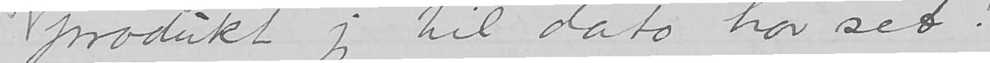produktet jeg til dato har set!
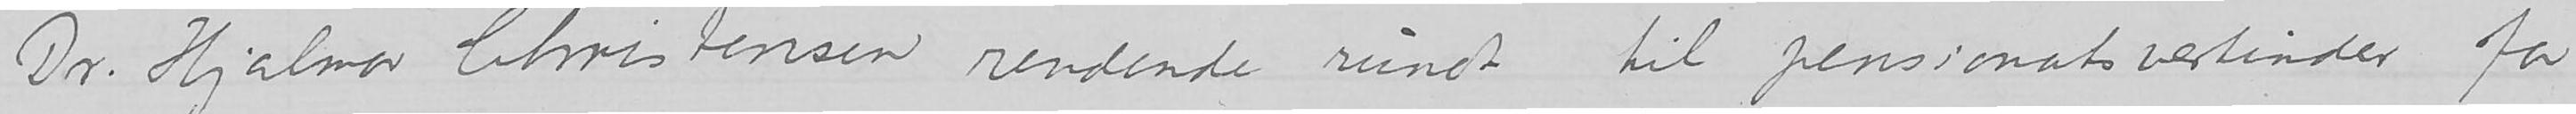Dr. Hjalmar Christensen rendende rundt til pensionatsvertinder for
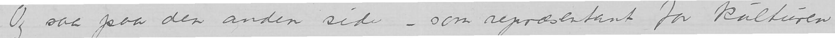Og saa paa den anden side – som repræsentant for kulturen
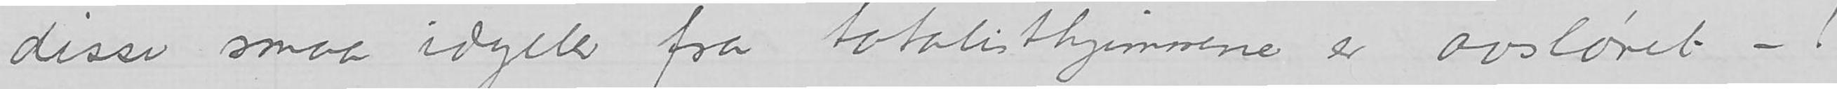disse smaa idyller fra totalisthjemmene er avsløret –!
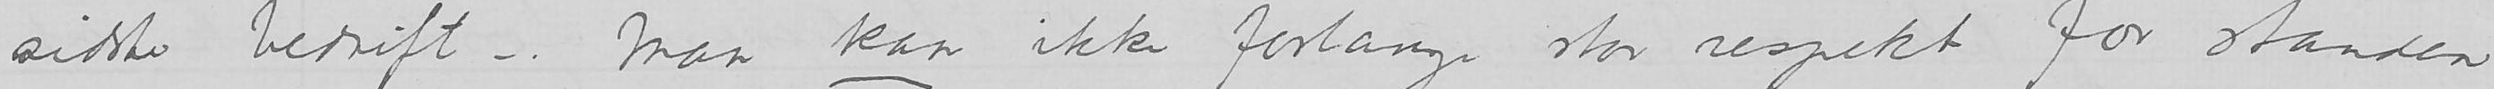sidste bedrift –. Man kan ikke forlange stor respekt for standen
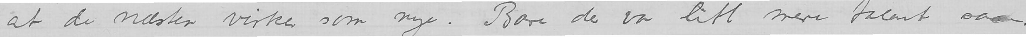at de næsten virker som nye. Bare der var litt mere talent saan.
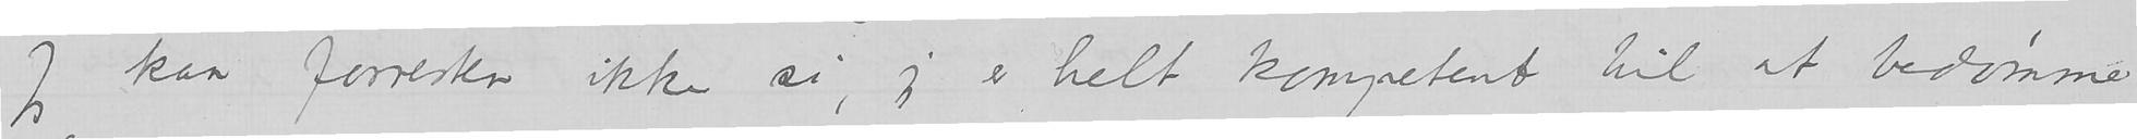Jeg kan forresten ikke si, jeg er helt kompetent til at bedømme
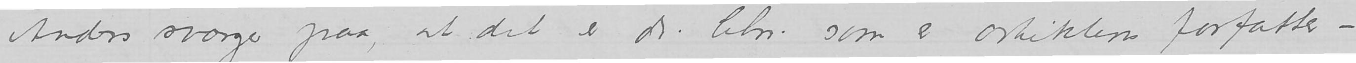Anders sværger paa, at det er dr. Chr. som er artiklens forfatter –
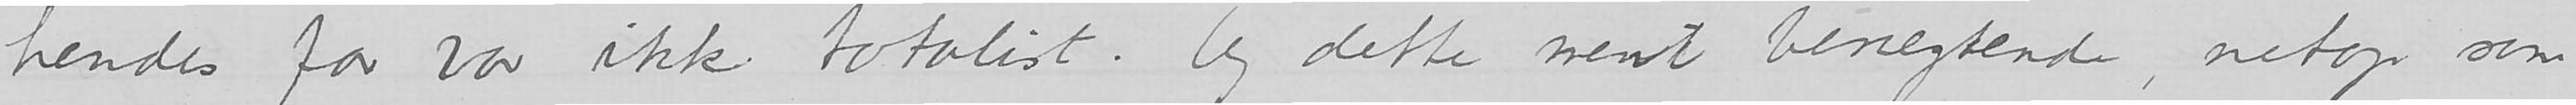hendes far var ikke totalist. Og dette ment benegtende, netop som
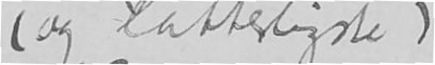(og latterligste)
